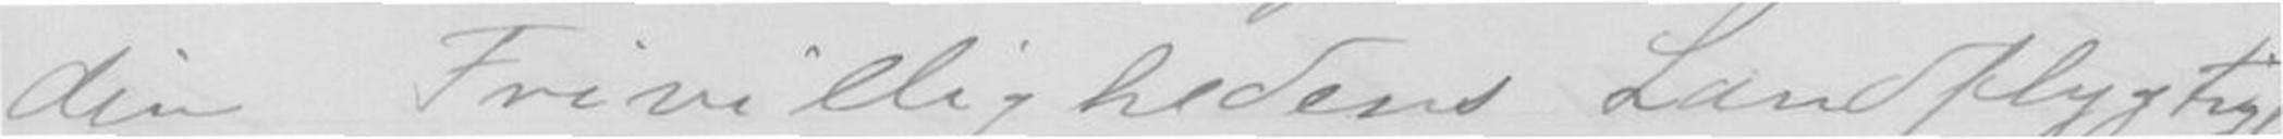din Frivillighedens Landflygtigheden?
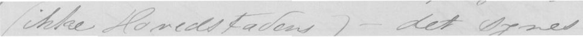(ikke hovedstadens) - det synes
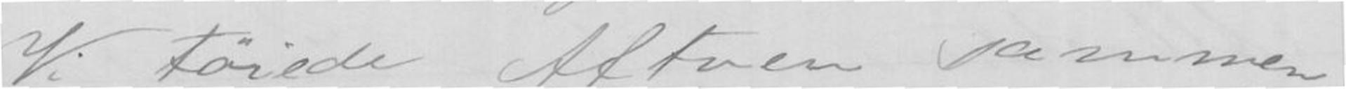Vi tøiede Aftnen sammen
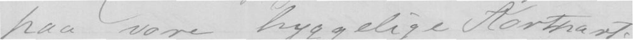paa vore hyggelige Kortpartier
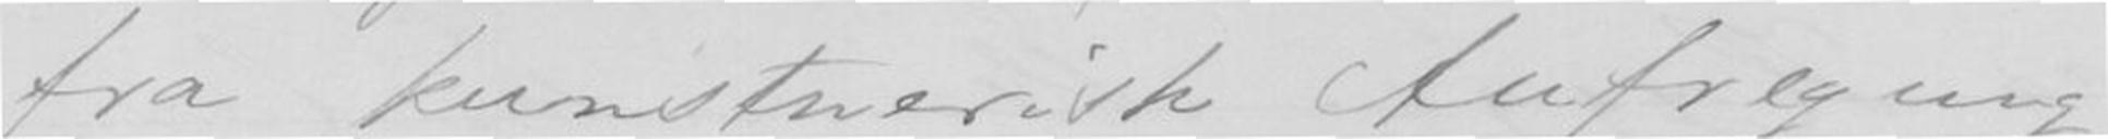fra kunstnerisk Aufregung
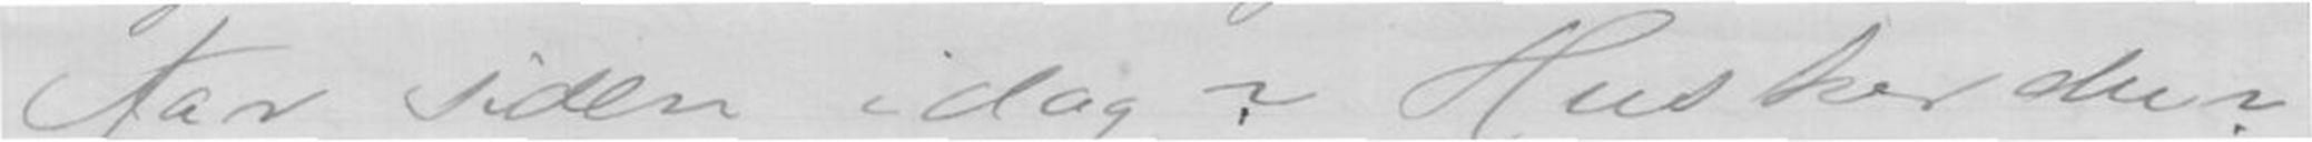Aar siden idag? Husker du?
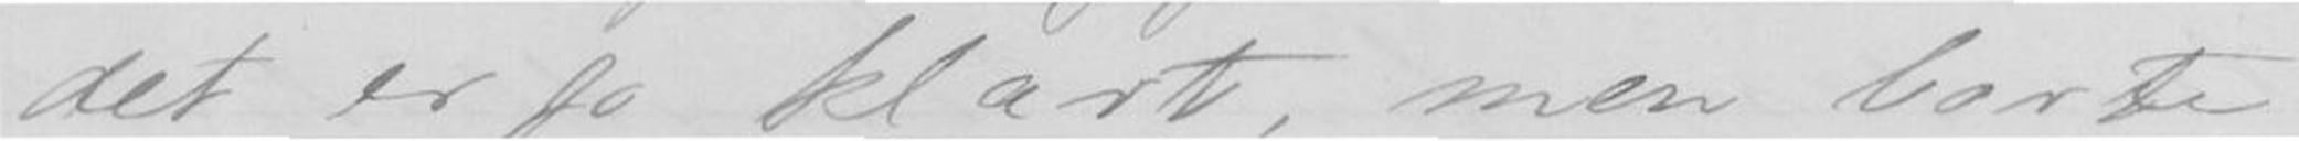det er jo klart, men borte
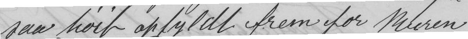saa høit opfyldt frem for Muren
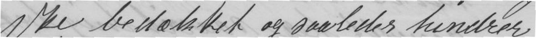ske bedækket og saaledes hindrer
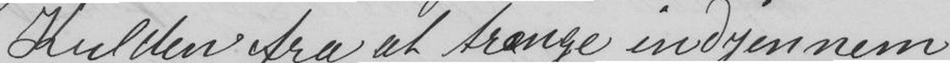Kulden fra at trænge indjennem
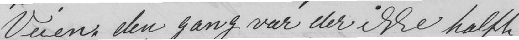Veien, den gang var der ikke halft
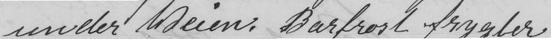under Veien. Barfrost frygter
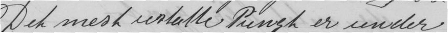Det mest utsatte Pungt er under
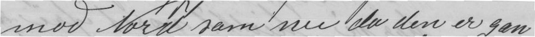mod Nord som nu da den er gan-
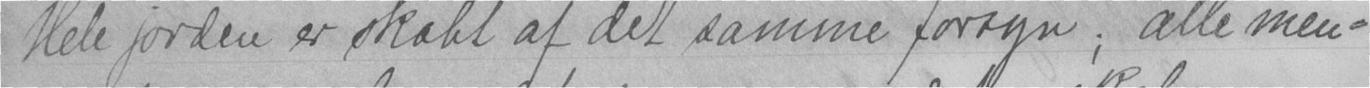Hele jorden er skabt af det samme forsyn; alle men-
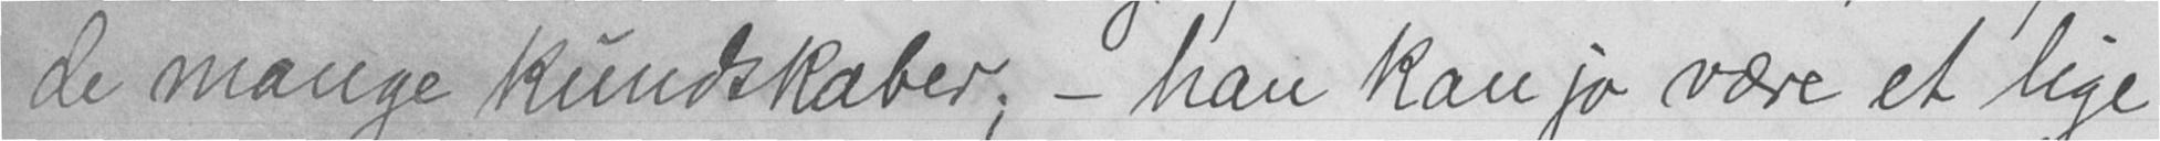de mange kundskaber; - han kan jo være et lige
